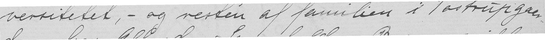versitetet, - og resten af familien i Tostrupgaar-
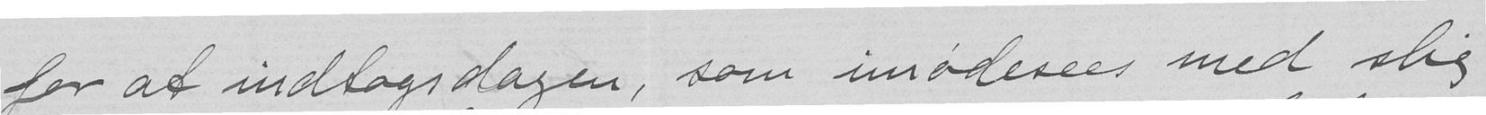for at indtagsdagen, som imødesees med slig
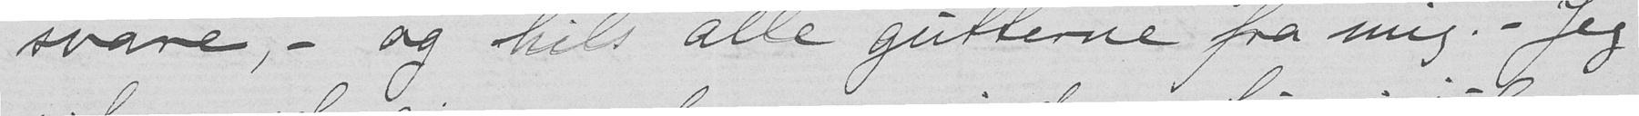svare, - og hils alle gutterne fra mig. - Jeg
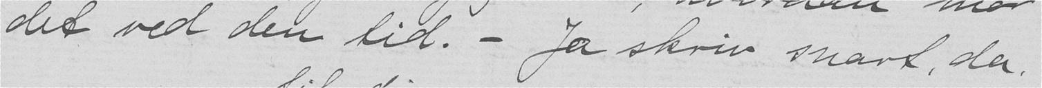det ved den tid. - Ja skriv snart, da,
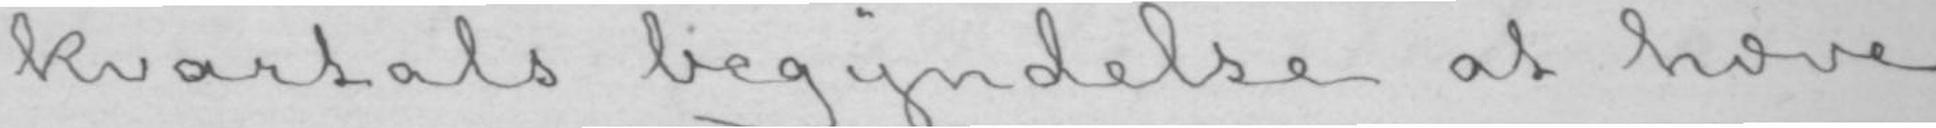kvartals begyndelse at hæve
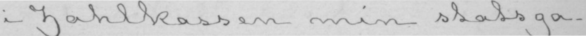i Zahlkassen min statsga-
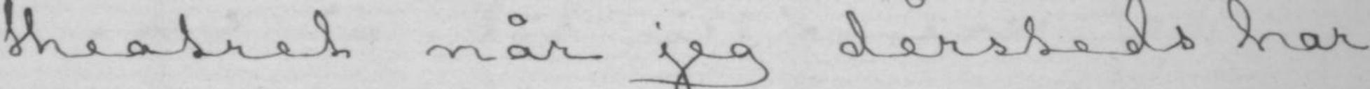theatret når jeg dersteds har
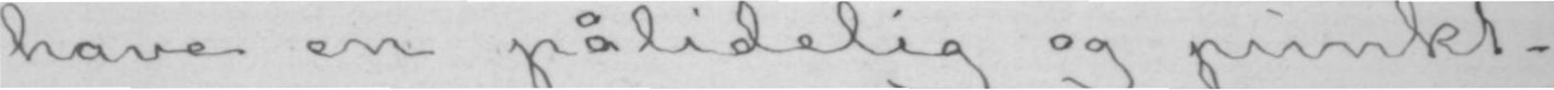have en pålidelig og punkt-
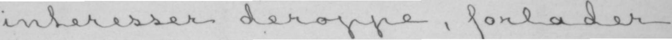interesser deroppe, forlader
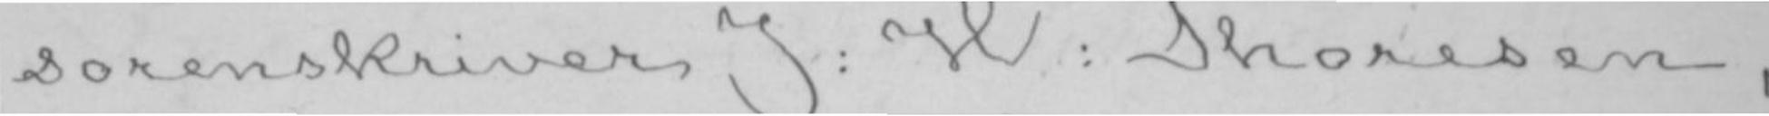sorenskriver J: H: Thoresen,
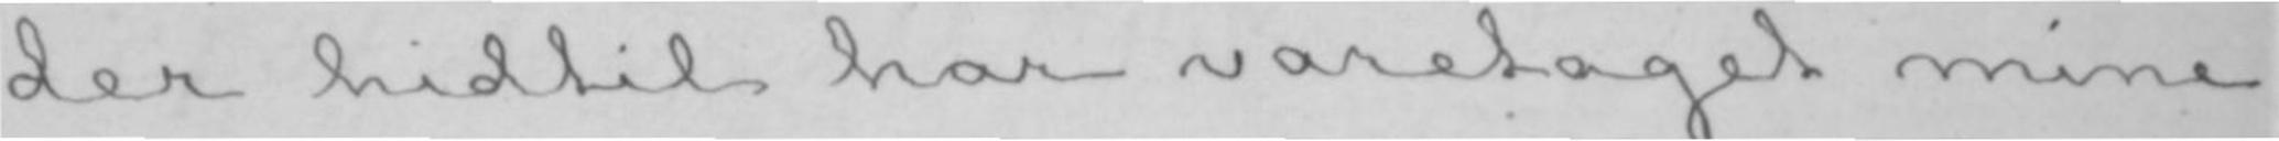der hidtil har varetaget mine
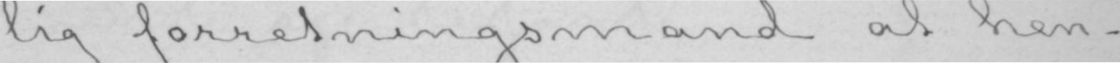lig forretningsmand at hen-
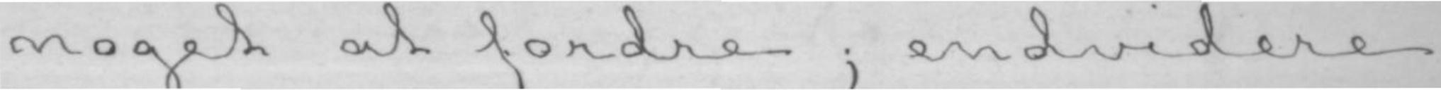noget at fordre; endvidere
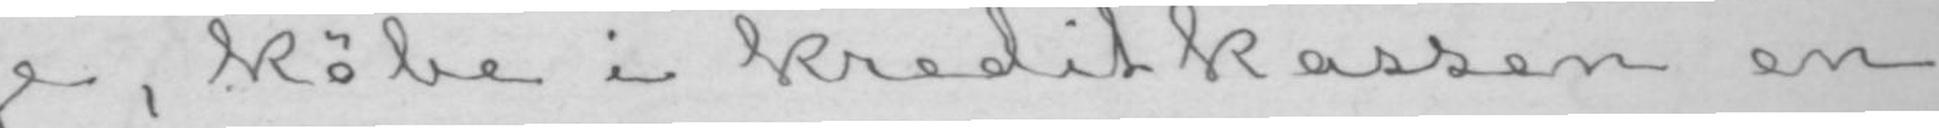ge, købe i kreditkassen en
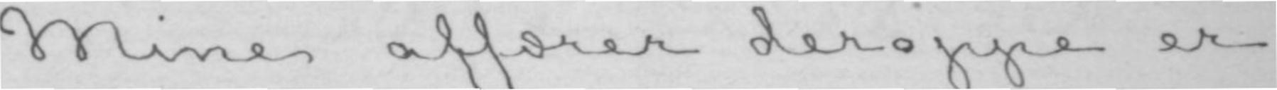Mine affærer deroppe er
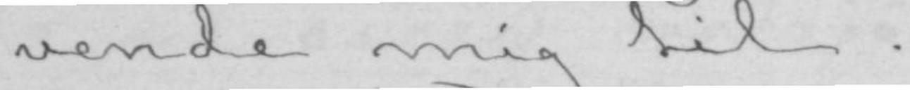vende mig til.
